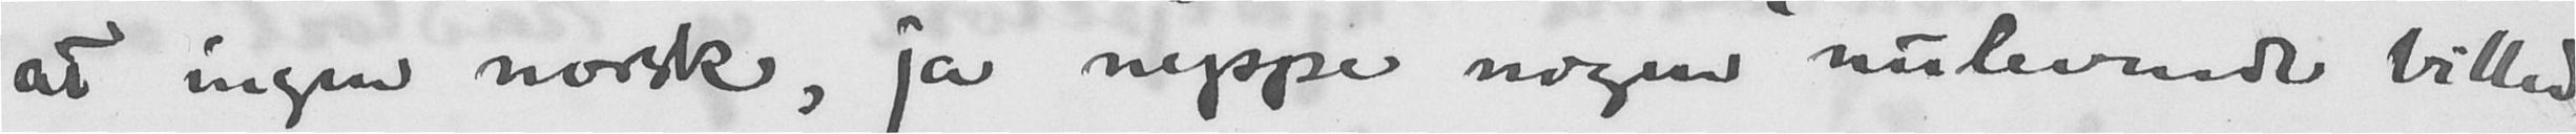at ingen norsk, ja neppe nogen nulevende billed-
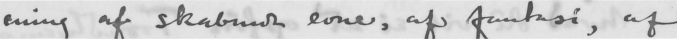ening af skabende evne, af fantasi, af
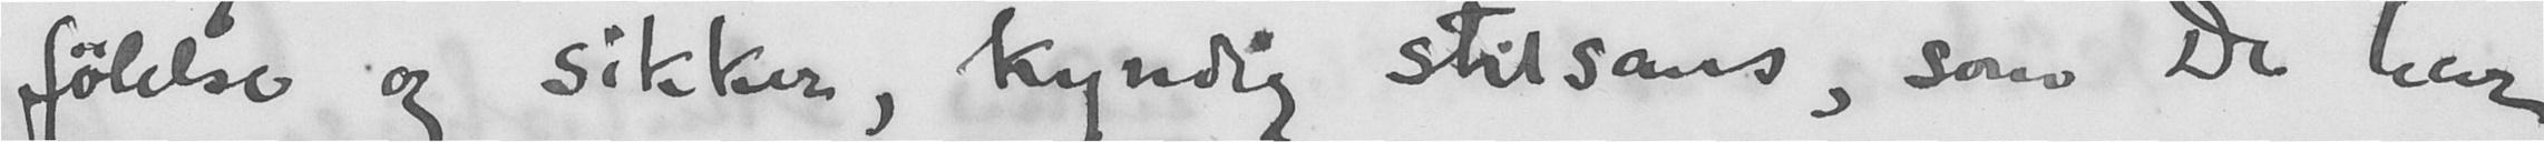følelse og sikker, kyndig stilsans, som De har
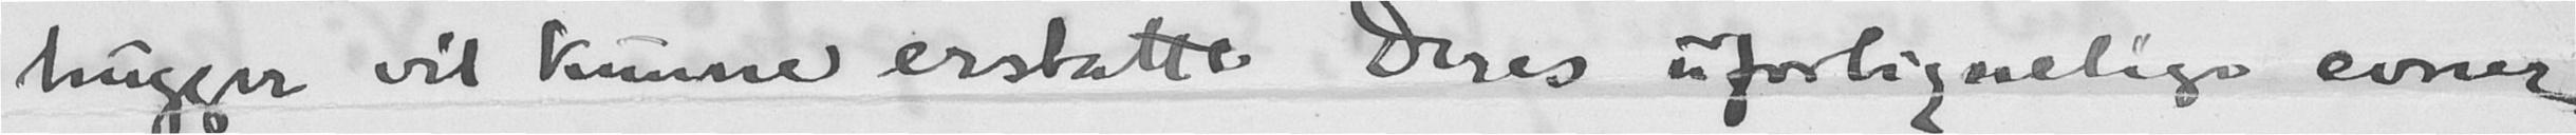hugger vil kunne erstatte Deres uforlignelige evner
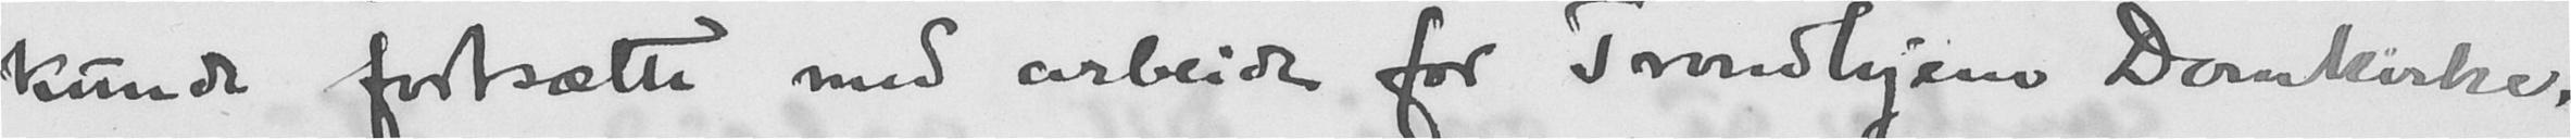kunde fortsætte med arbeidet for Trondhjem Domkirke.
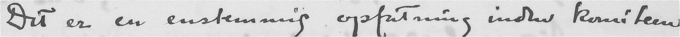Det er en enstemmig opfatning inden komiteen
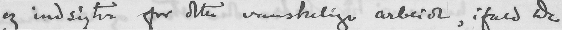og indsigter for dette vanskelige arbeide, ifald De
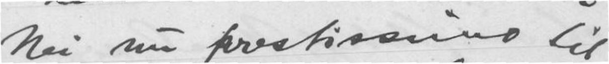Nei nu prestissimo til
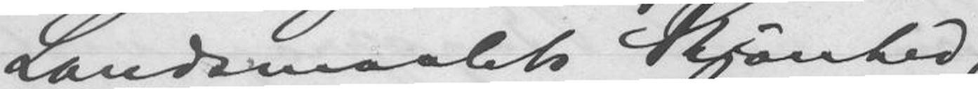Landsmaalets Skjønhed,
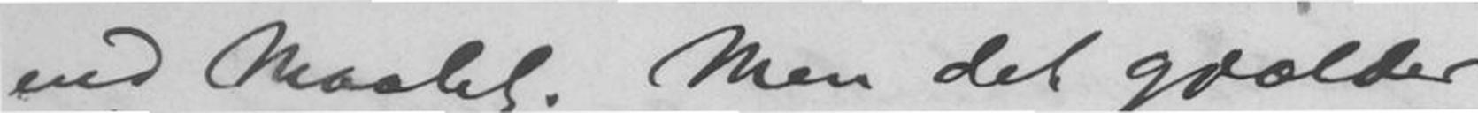end Maalet. Men det gjælder
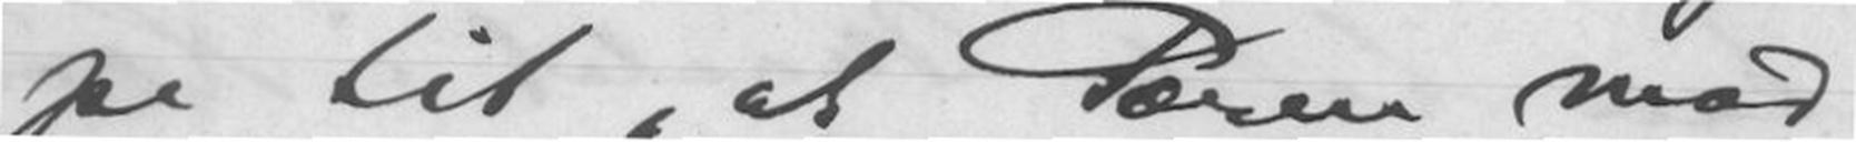pe til, at Pæren mod-
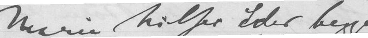Marie hilser Eder begge
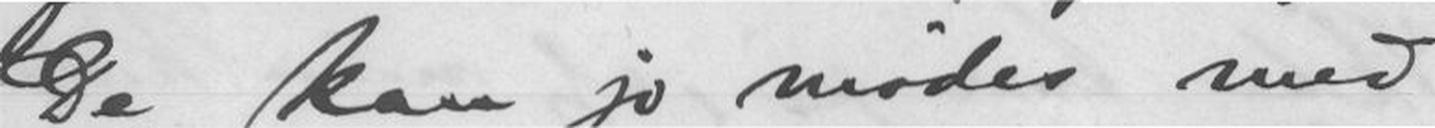De kan jo mødes med
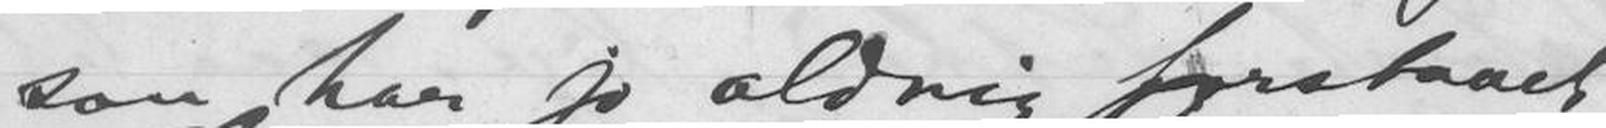son har jo aldrig forestaaet
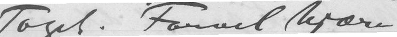Toget. Farvel kjære
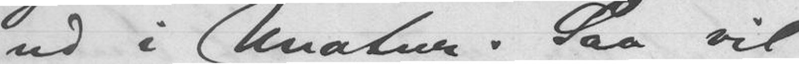ud i Unatur. Saa vil
